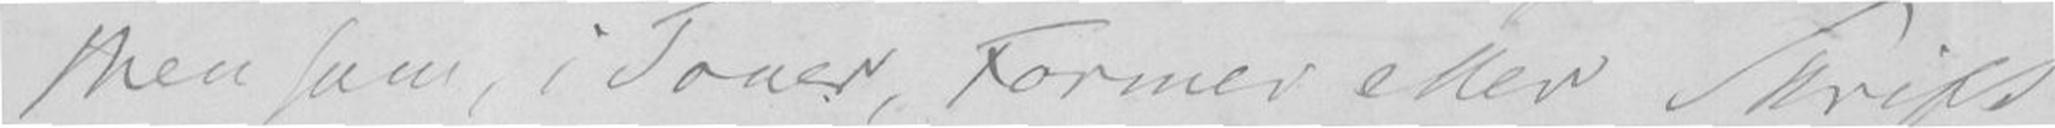Men som, i Toner, Former eller Skrift
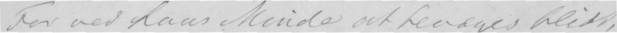For ved hans Minde at bevæges blidt,
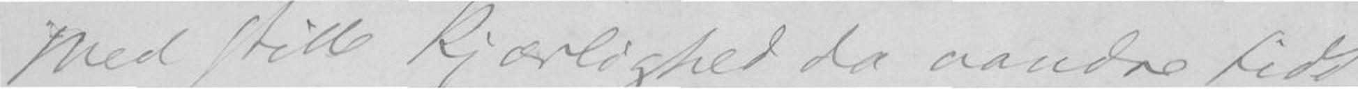Med stille Kjærlighed da vandre tidt
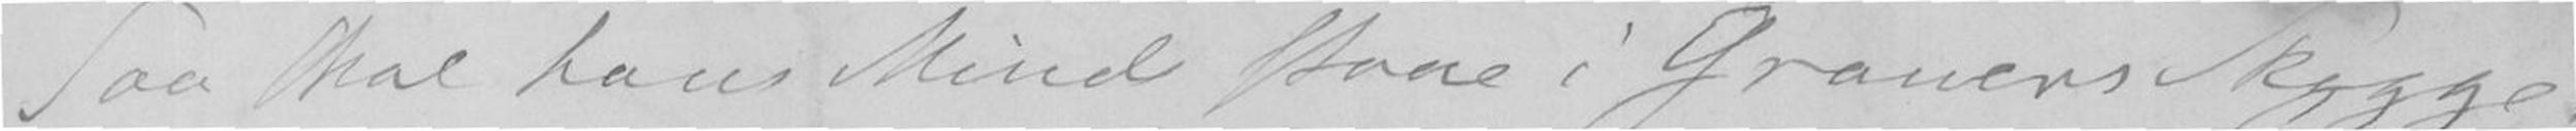Saa skal hans Minde staae i Graners Skygge.
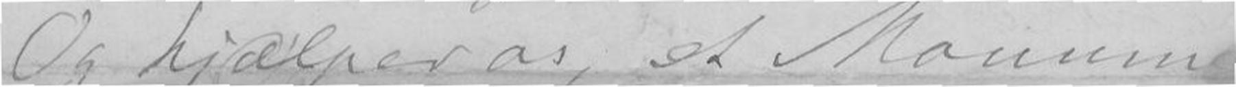Og hjælper os, at Monum
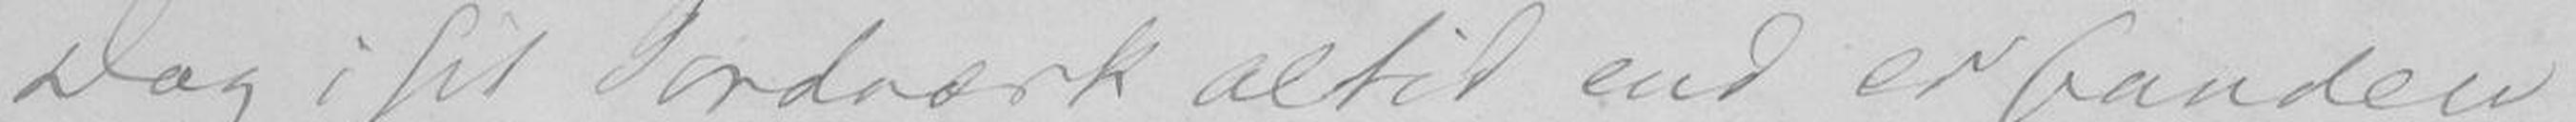Dag i sit Jordværk altid end er bunden.
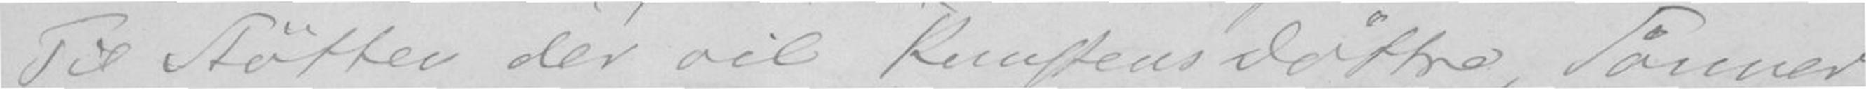Til Støtten der vil Kunstens Døttre, Sønner
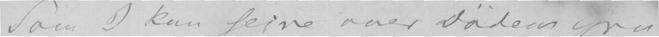Som I kan feire over Dødens Gru,
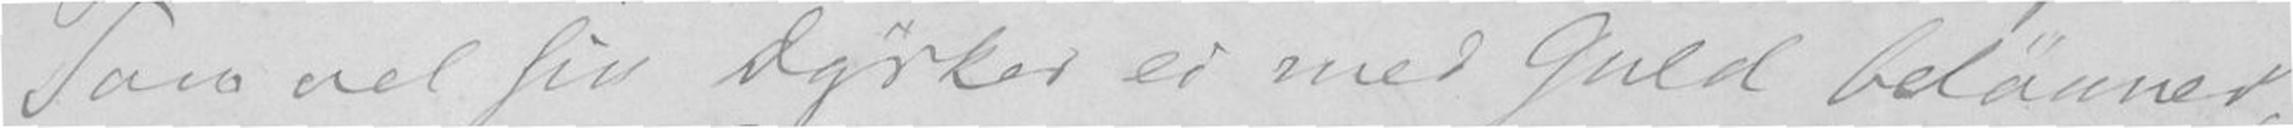Som vel sin Dyrker ei med Guld belønner,
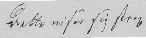Dette viser sig strax
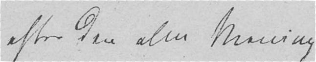efter den alm Mening
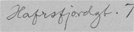Hafrsfjordgt. 7
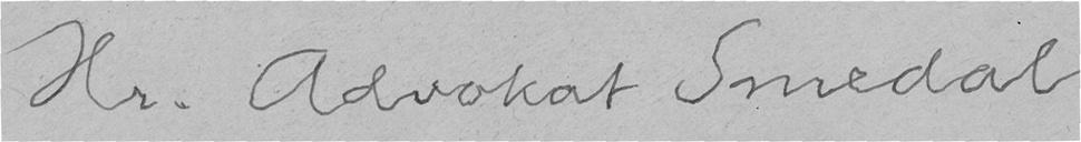Hr. Advokat Smedal
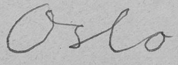Oslo
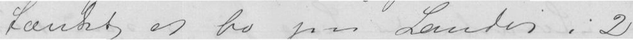tænkt at bo paa Landet i 2
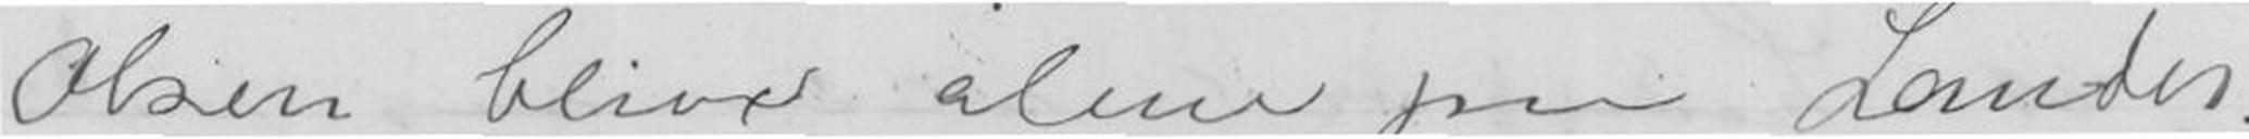Olsen blive alene paa Landet.
